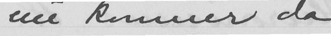nu kommer da
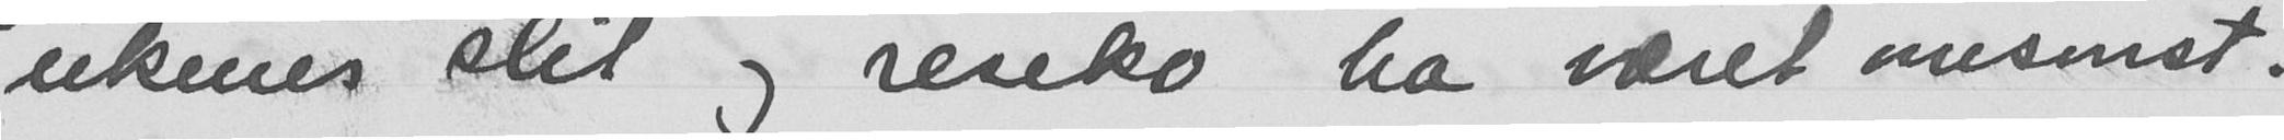ukenes slit og resiko ha veret omsonst.
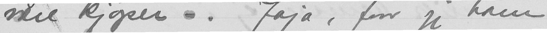være kjøper -. Jaja, faar jeg ham
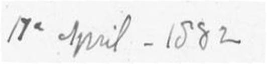17de April - 1882
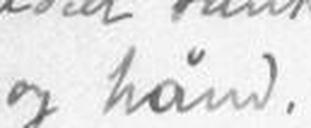og hånd.
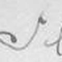Til
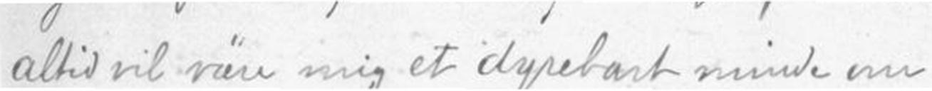altid vil väre mig et dyrebart minde om
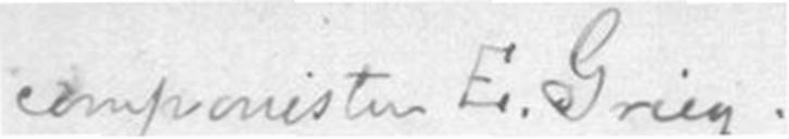componisten E. Grieg!
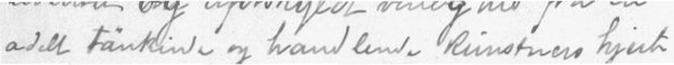adelt tänkinde og handlende kunstners hjerte
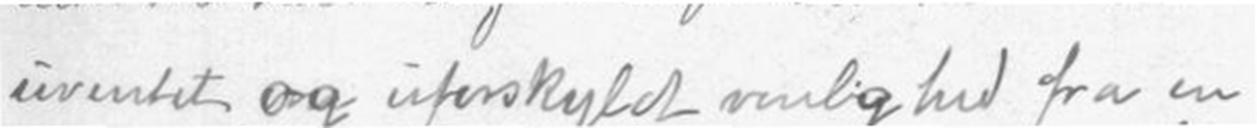uventet og uforskyldt venlighed fra en
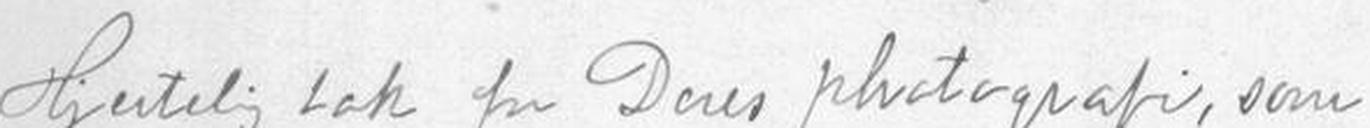Hjertelig tak for Deres photografi, som
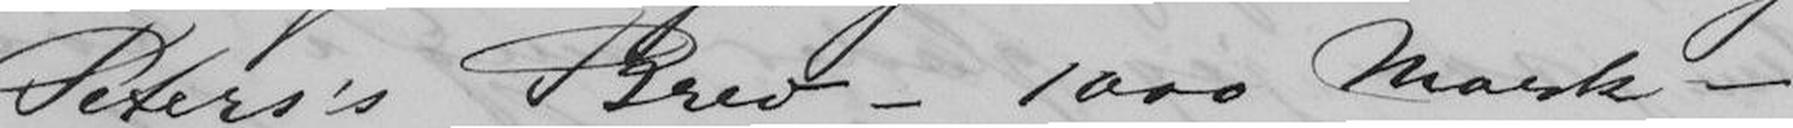Peters's Brev - 1000 Mark -
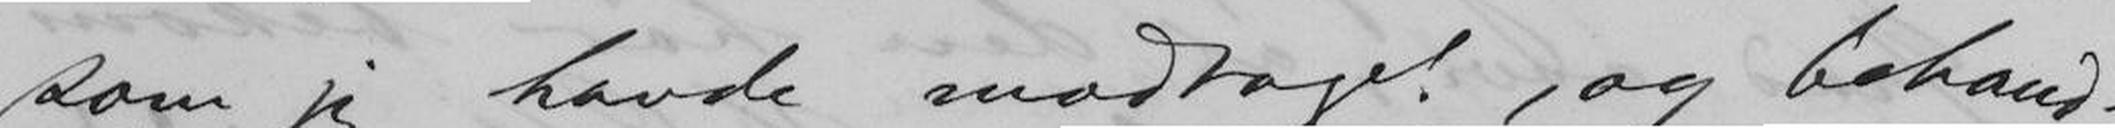som jeg havde modtaget, og behand-
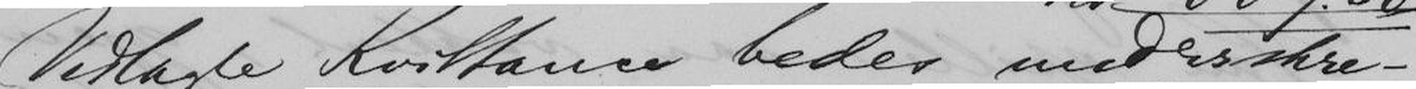Vedlagte Kvittance bedes underskre-
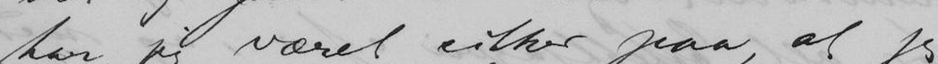har jeg været sikker paa, at jeg
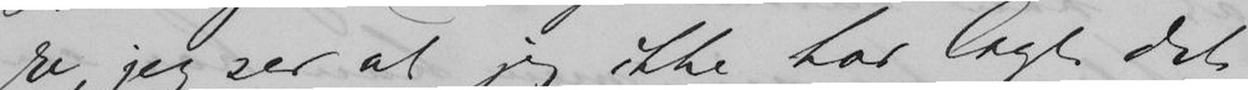re, jeg ser at jeg ikke har lagt det
